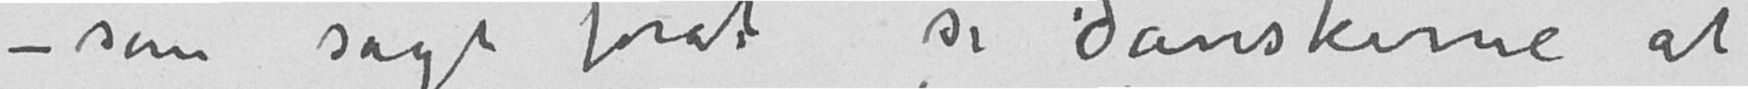– som sagt forat si danskerne at
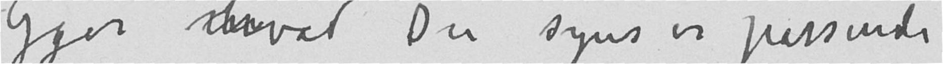Gjør hvad Du syns er passende
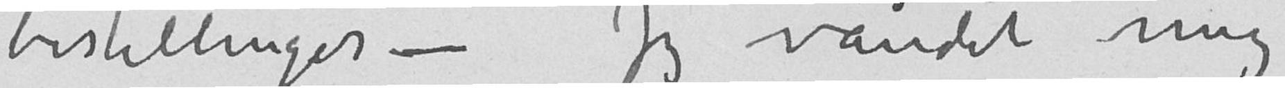bestillinger – Jeg våndet mig
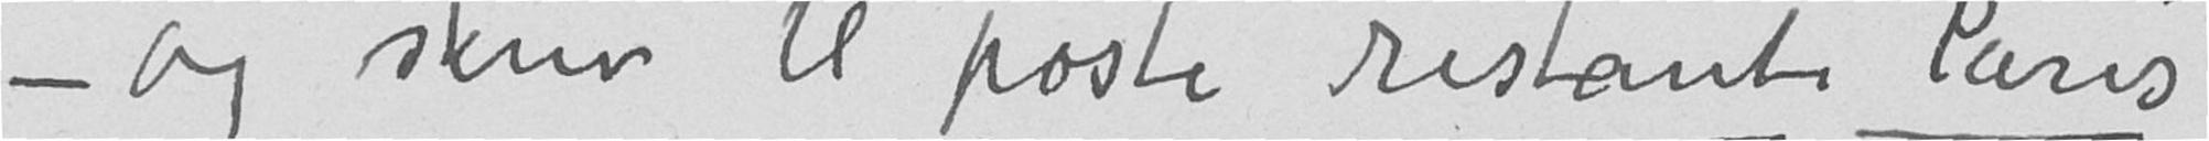– og skriv til poste restante Paris
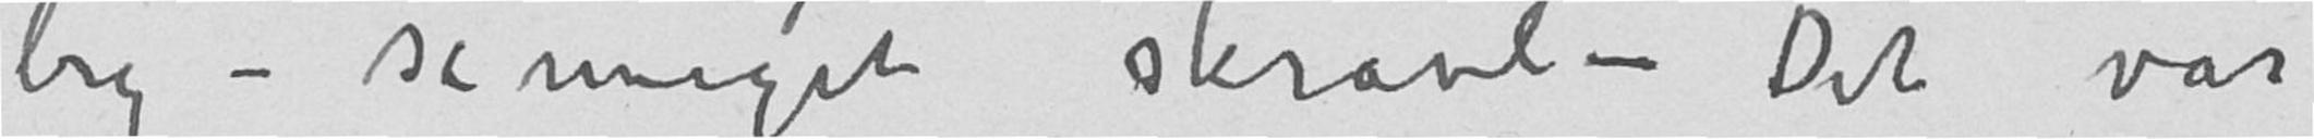by – så meget skravl – Det var
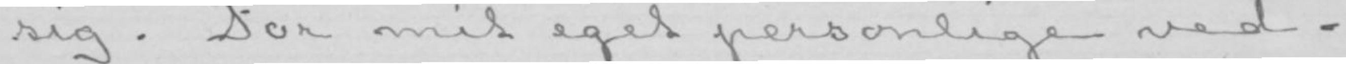sig. For mit eget personlige ved-
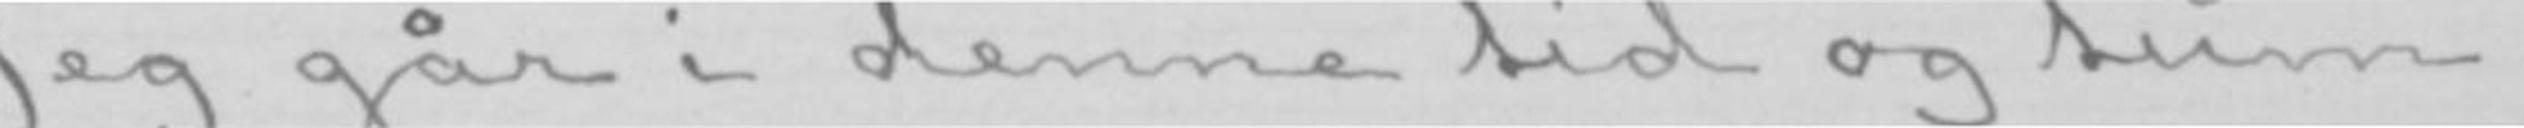Jeg går i denne tid og tum-
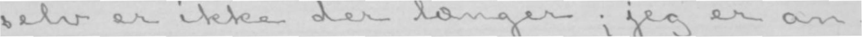selv er ikke der længer; jeg er an-
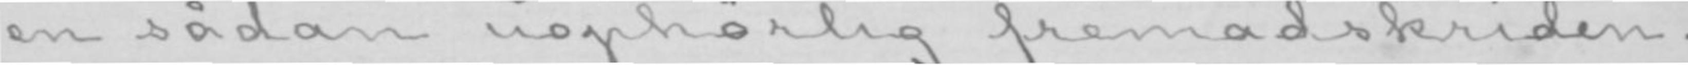en sådan uophørlig fremadskriden.
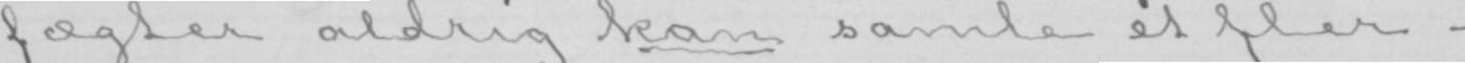fægter aldrig kan samle et fler-
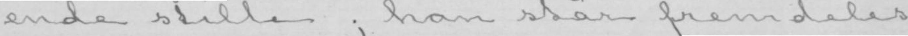ende stille; han står fremdeles
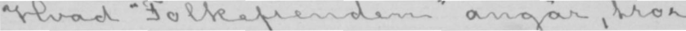Hvad «Folkefienden» angår, tror
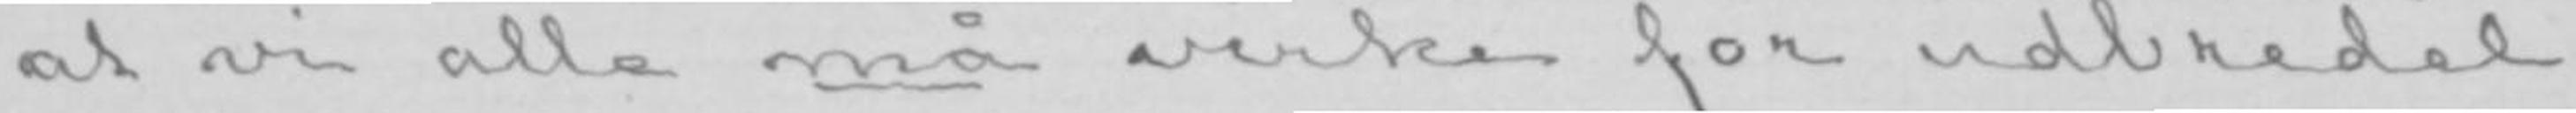at vi alle må virke for udbredel-
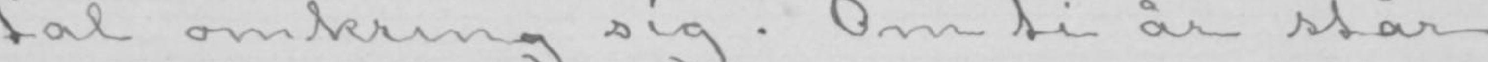tal omkring sig. Om ti år står
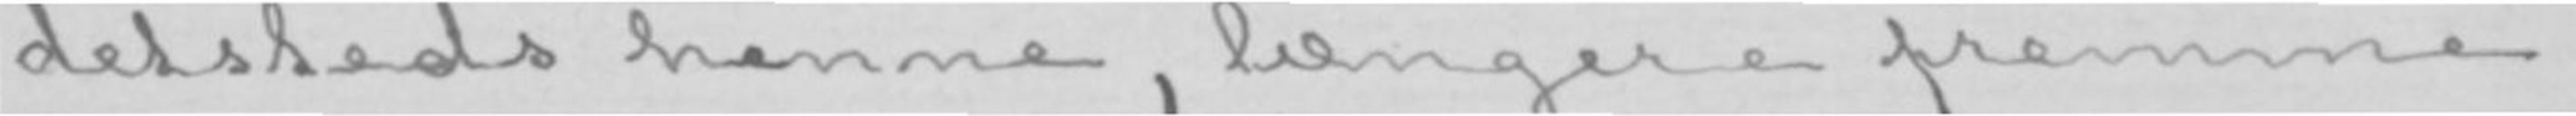detsteds henne, længere fremme,
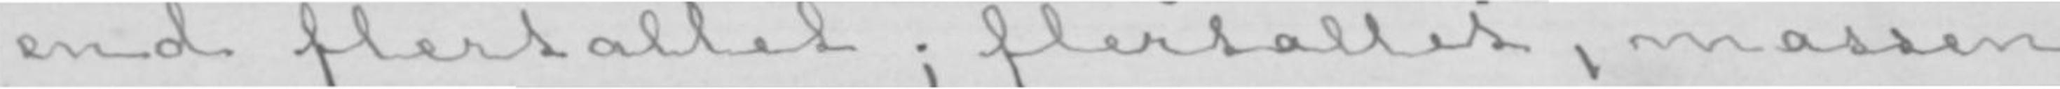end flertallet; flertallet, massen,
